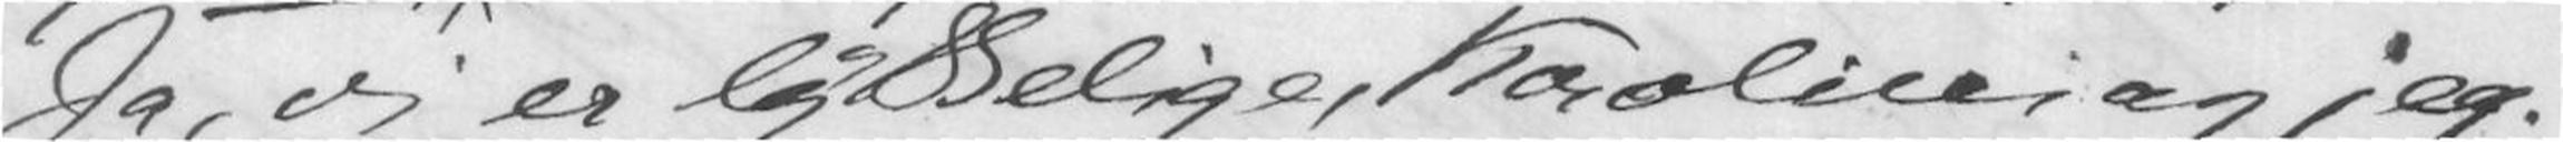Ja, vi er lykkelige, Karoline og jeg.
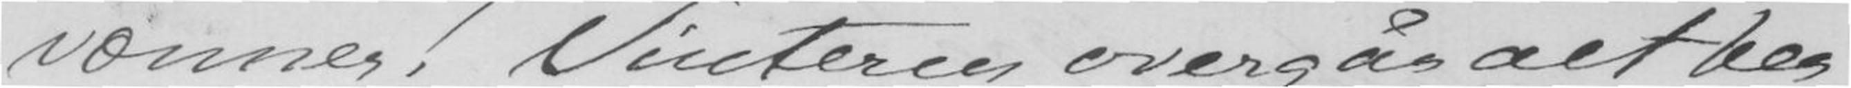vænner! Vinteren overgår alt her
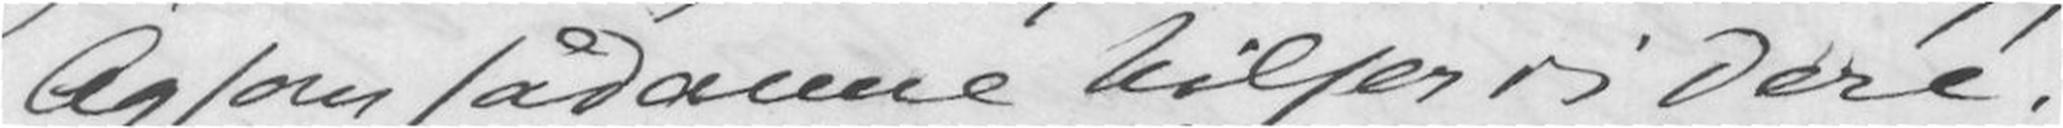Og som sådanne hilser vi Dere!
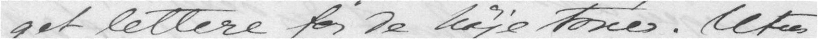get lettere for de høje toner. Uten
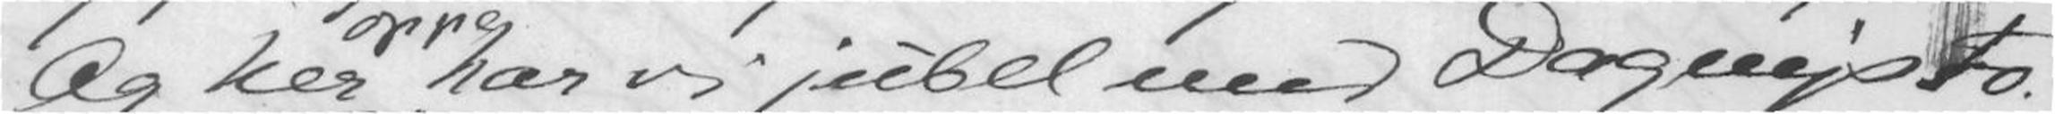Og her har vi jubel med Dagny's to.
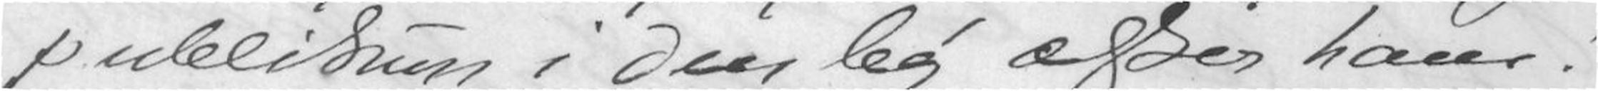publikum i den leg ælsker ham!
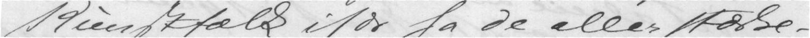Kunstfolk især sa de aller stærke-
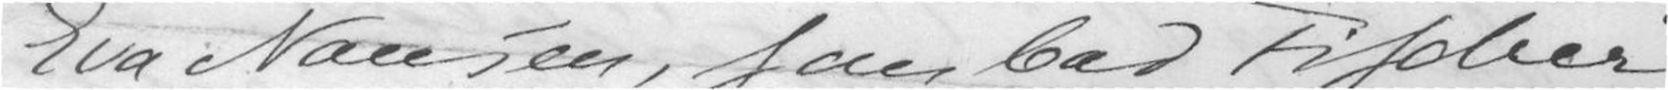Eva Nansen, som bad Fischer
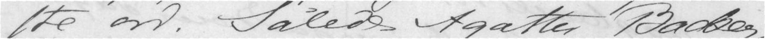ste ord. Således Agathe Backer,
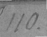110.
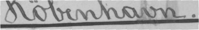København.
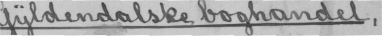Gyldendalske boghandel,
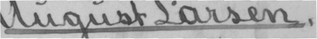August Larsen,
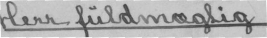Herr fuldmægtig
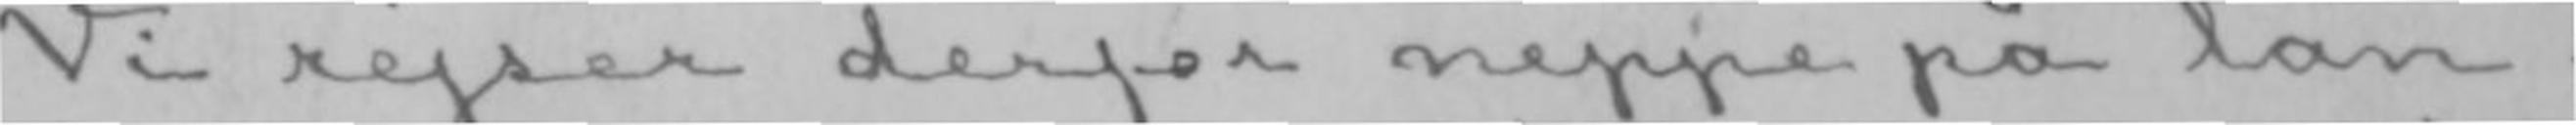Vi rejser derfor neppe på lan-
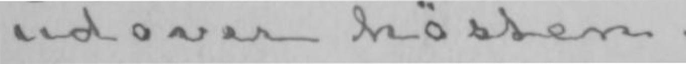udover høsten.
